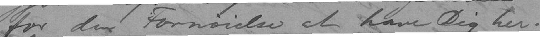for den Fornøielse at have Dig her.
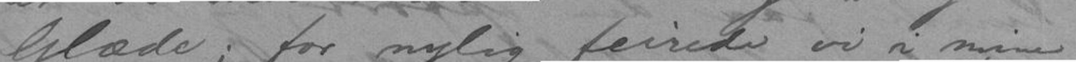Glæde; for nylig feirede vi i mine
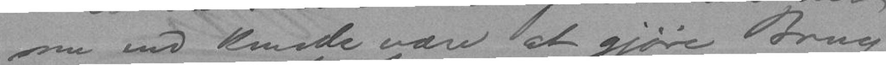nu end kunde være at gjøre Brug
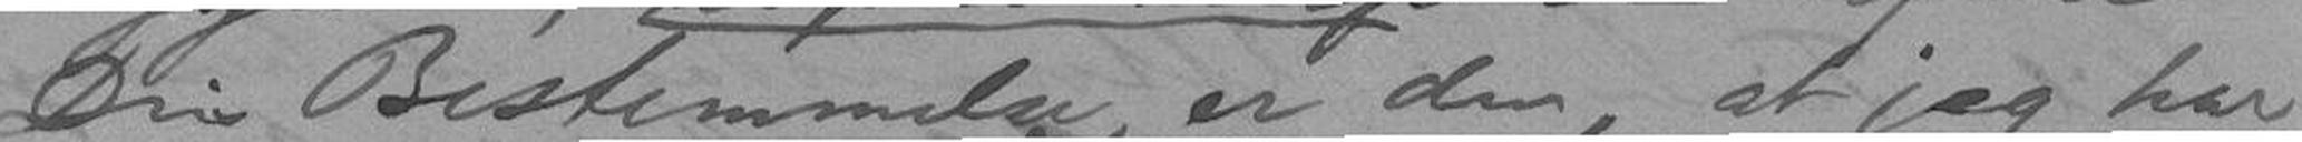Din Bestemmelse, er den, at jeg har
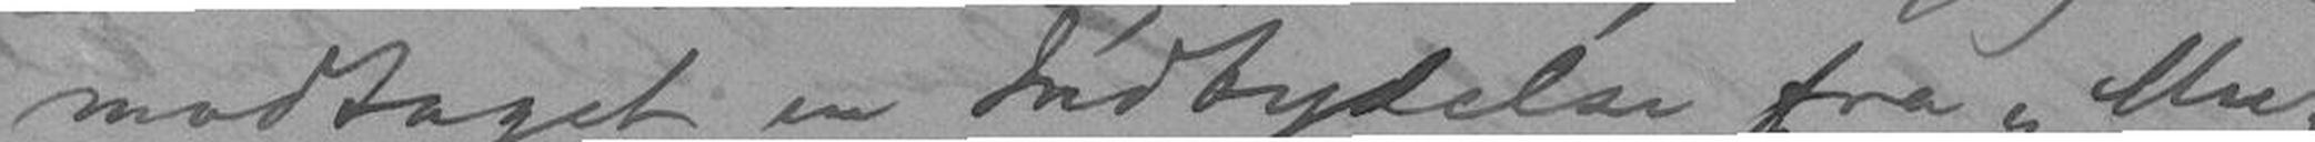modtaget en Indbydelse fra Mu-
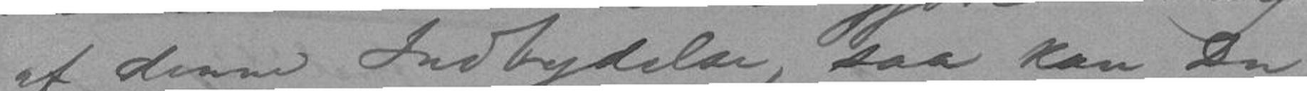af denne Indbydelse, saa kan Du
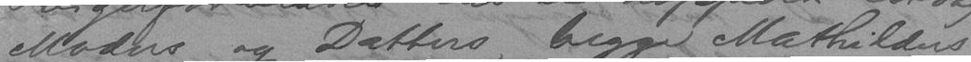Moders og Datters, begge Mathilders
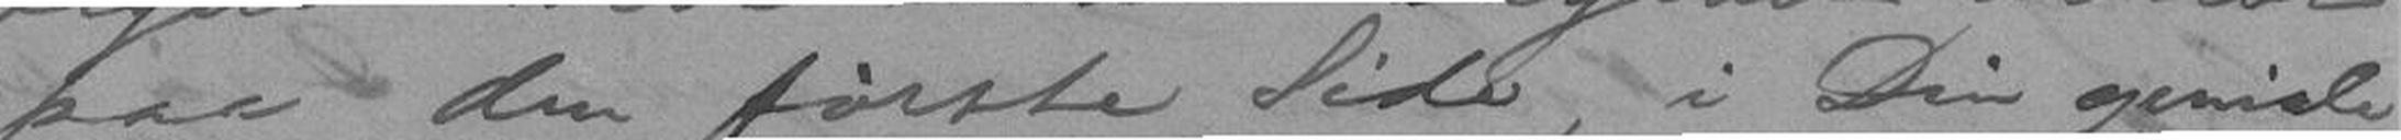paa den første Side, i Din geniale
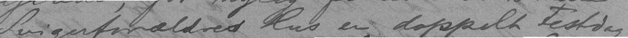Svigerforældres Hus en doppelt Festdag,
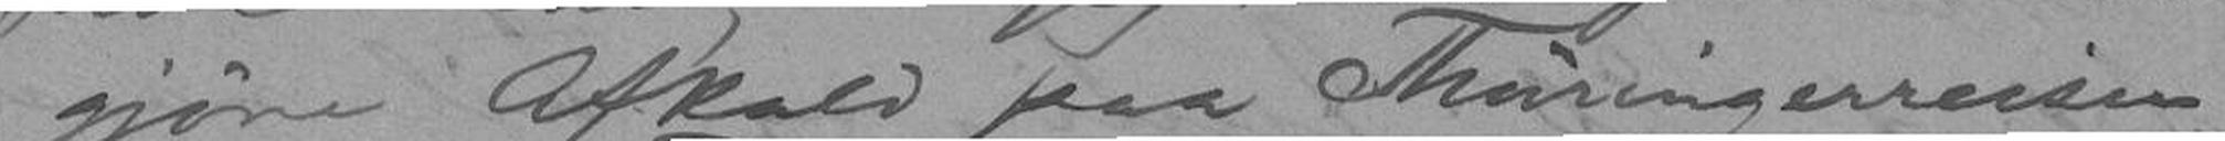gjøre Afkald paa Thüringerreisen
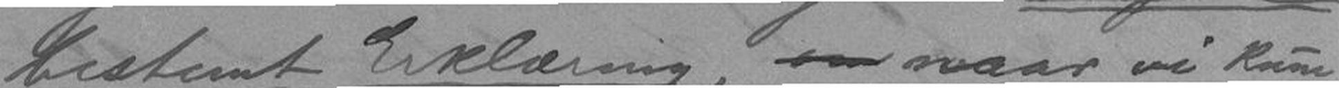bestemt Erklæring, naar vi kunne
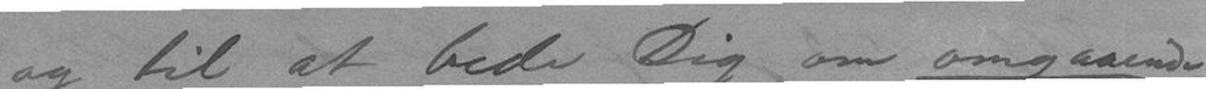og til at bede Dig om omgaaende
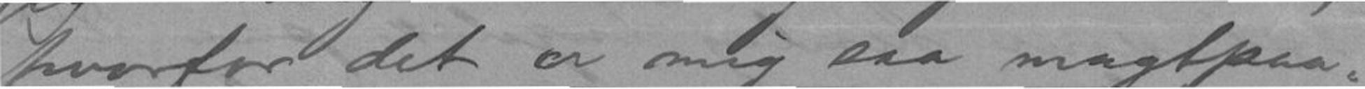hvorfor det er mig saa magtpaa-
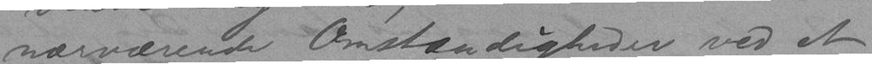nærværende Omstændigheder ved at
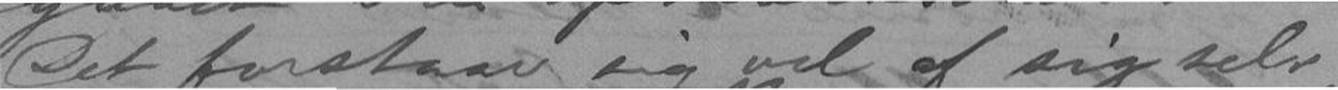Det forstaar sig vel af sig selv,
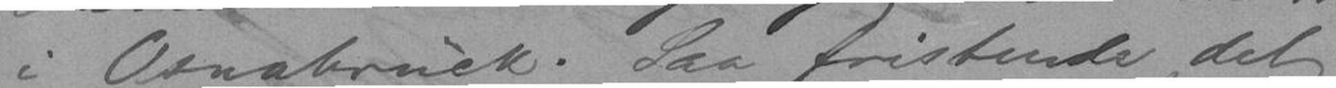i Osnabrück. Saa fristende det
